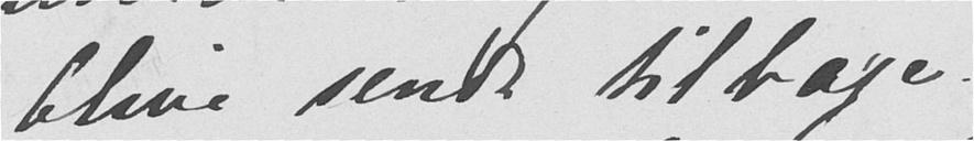blive sendt tilbage.
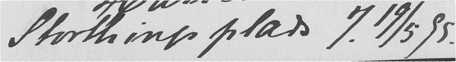Storthingsplads 7. 19/5 95.
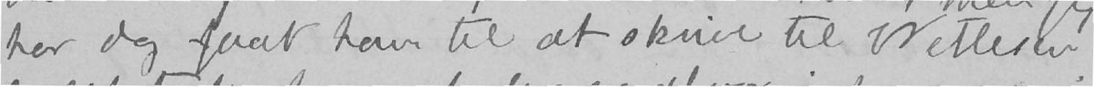har dog faat ham til at skrive til Wetlesen
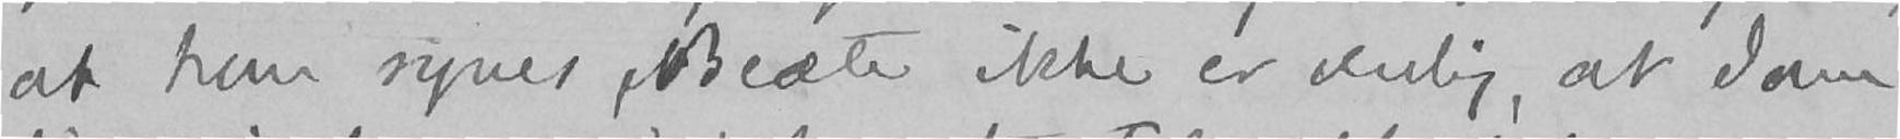at hun synes Beate ikke er venlig, at Jane
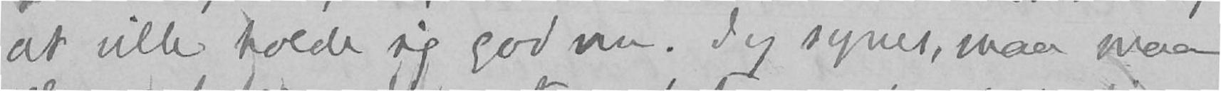at ville holde sig god nu. Jeg synes, man maa
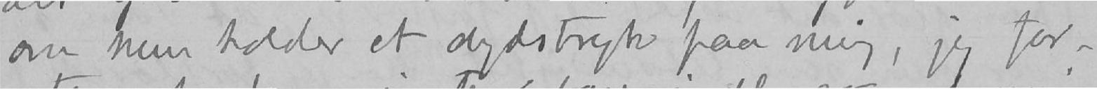om hun holdet et dydstryk paa mig, jeg for-
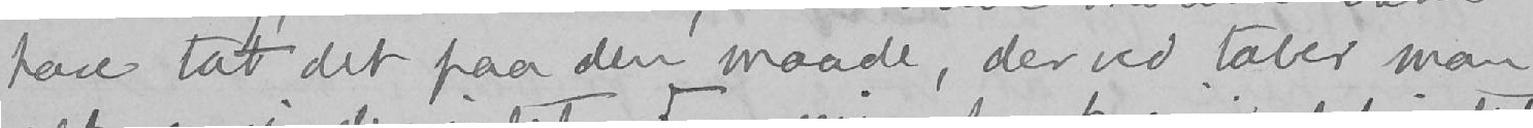have tat det paa den maaden, derved taber man
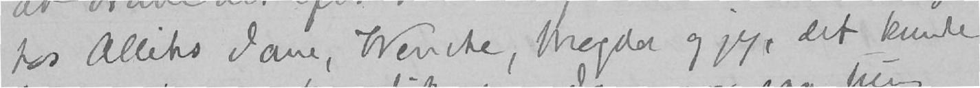hos Alliks Jane, Wenche, Magda og jeg, det kunde
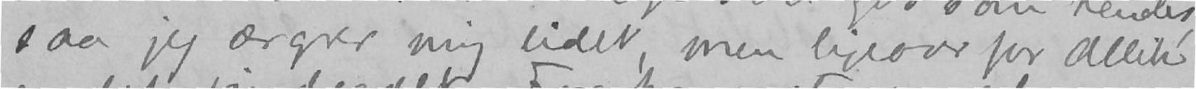saa jeg ærgrer mig lidet, men ligeover for Allik
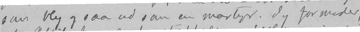som bly og saa ud som en martyr. Jeg formoder,
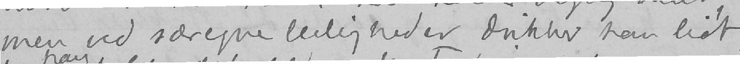men ved særegne leiligheder drikker han lidt,
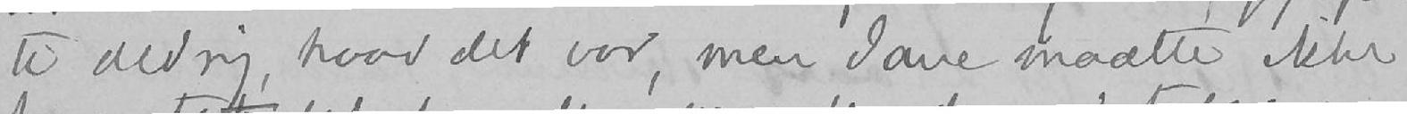te aldrig, hvad det var, men Jane maatte ikke
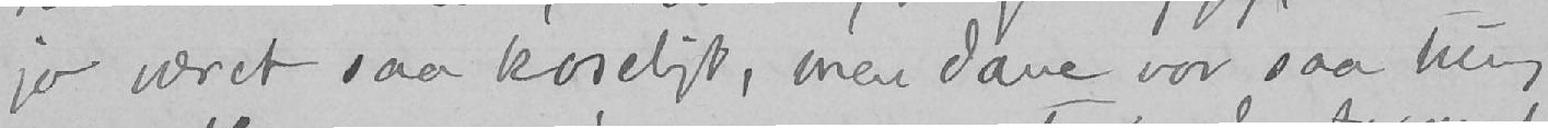jo været saa koseligt, men Jane var saa tung
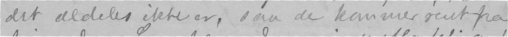det aldeles ikke er, saa de kommer rent fra
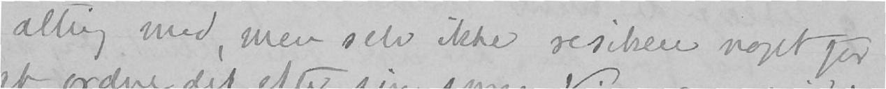alting med, men selv ikke resikere noget for
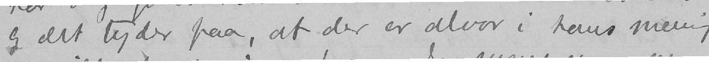og det tyder paa, at der er alvor i hans mening
